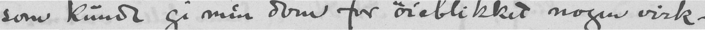som kunde gi min dom for øieblikket nogen virk-
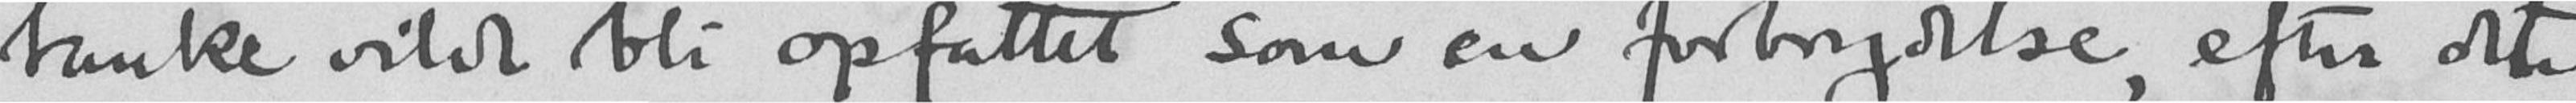tanke vilde bli opfattet som en forbrydelse, efter det
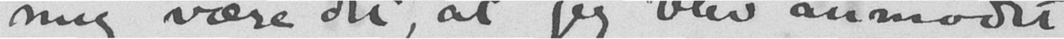mig være det, at jeg blev anmodet
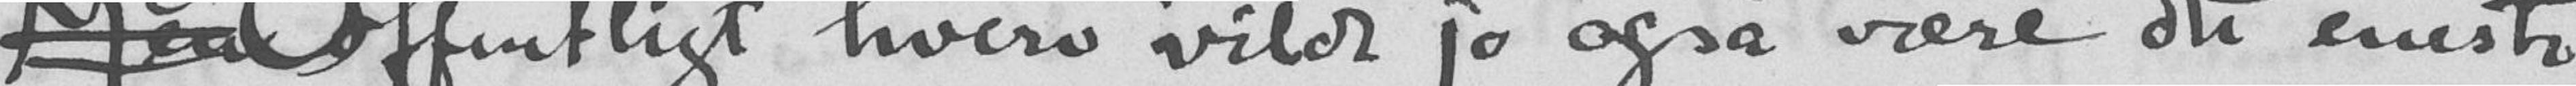Men "Offentligt hverv" vilde jo også være det eneste,
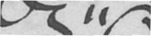Og
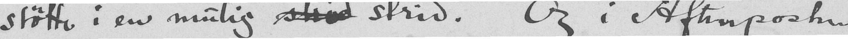støtte i en mulig strid strid. Og i "Aftenposten"
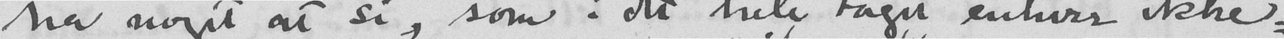ha noget at si, som i det hele taget enhver ikke =
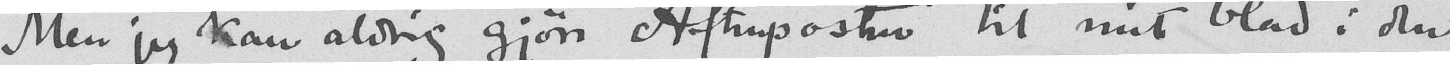Men jeg kan aldrig gjøre "Aftenposten" til mit blad i den
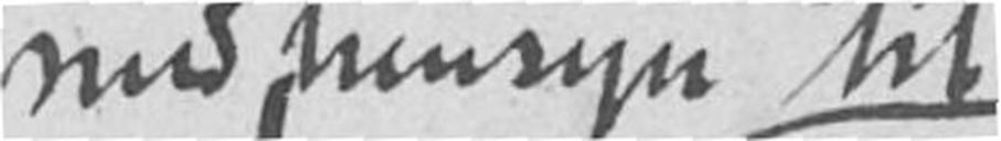med hensyn til
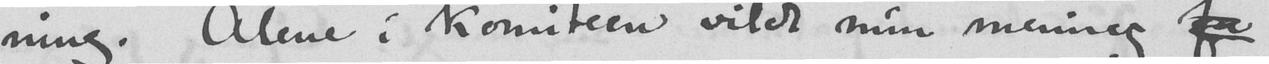ning. Alene i komiteen vilde min mening fa
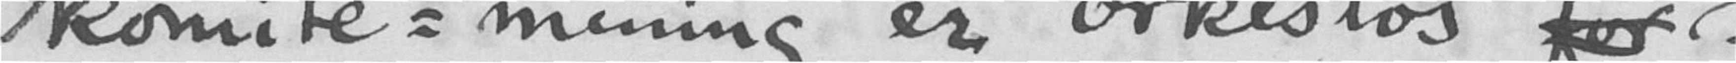komite = mening er ørkesløs for
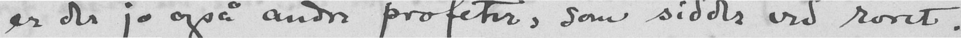er der jo også andre profeter, som sidder ved roret.
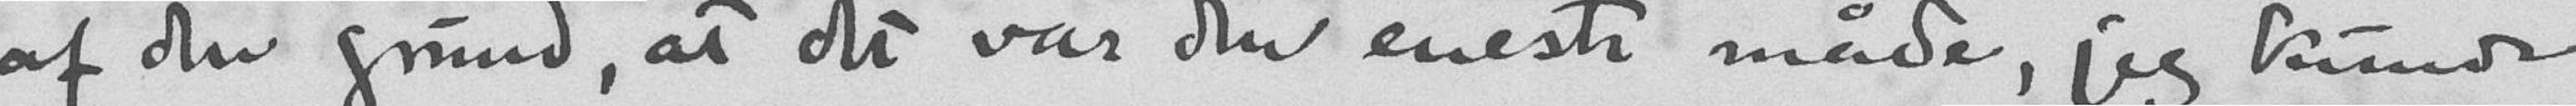af den grund, at det var den eneste måde, jeg kunde
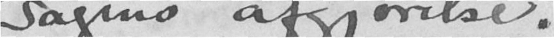sagens afgjørelse.
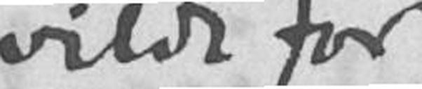vilde for
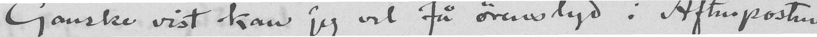Ganske vist kan jeg vel få ørenslyd i "Aftenposten".
